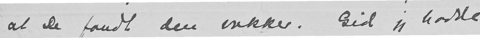at De fandt den vakker. Gid jeg hadde
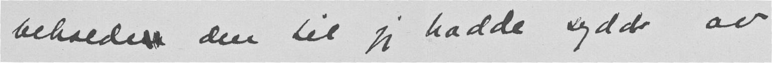beholde den til jeg hadde sydd av
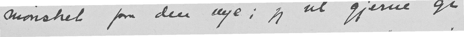mønstret paa den nye; jeg vil gjerne gi
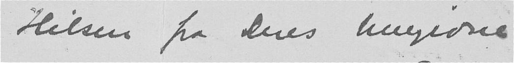Hilsen fra Deres hengivne
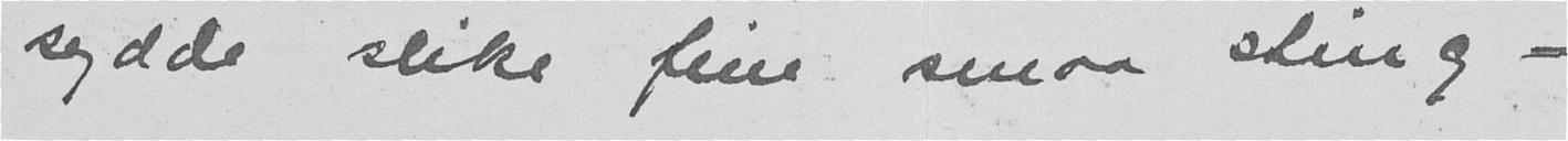sydde slike fine smaa sting -
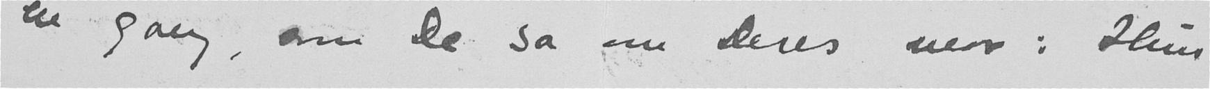en gang, som De sa om deres mor: Hun
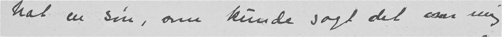hat en søn, som kunde sagt det om mig
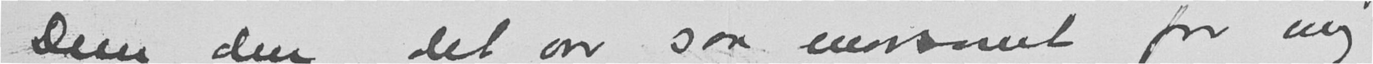Dem den, det var saa morsomt for mig
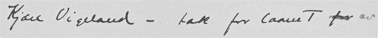Kjære Vigeland - tak for laanet for av
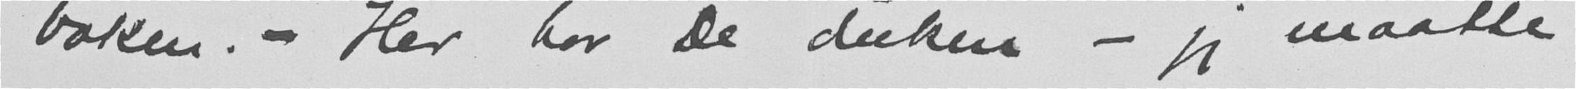boken.- Her har De duken - jeg maatte
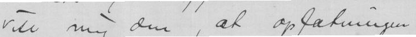vise mig om, at opfatningen
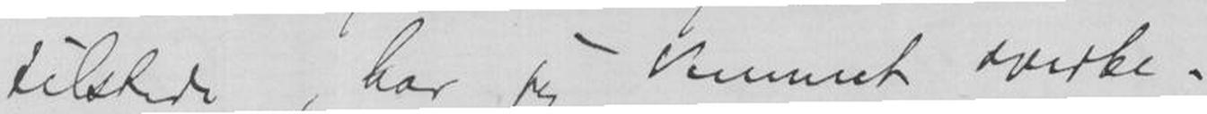tilstede, har jeg kunnet overbe-
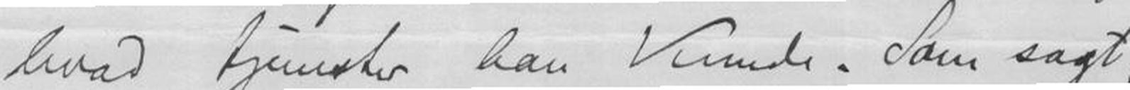hvad tjenester han kunde. Som sagt,
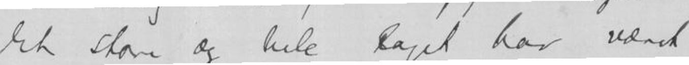i det store og hele taget har været
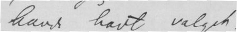havde havt valget.
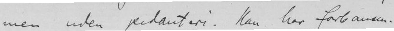men uden pedanteri. Han har forbausende
